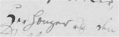Her hænger og den
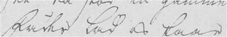Fader lad os faae
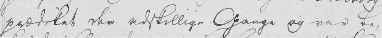prædiket der adskillige Gange og var be-
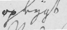opbygt
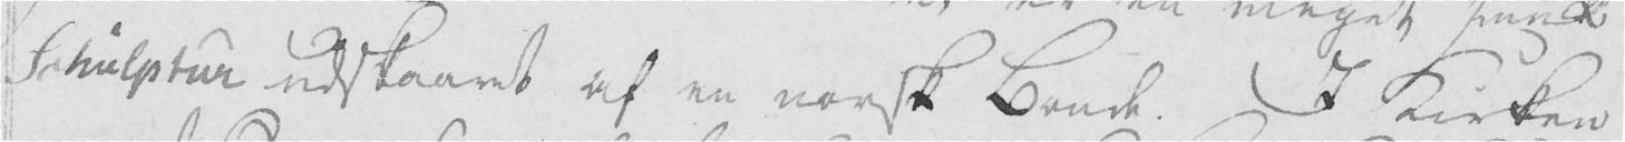Skulptur udskaaret af en norsk Bonde. I Kirken
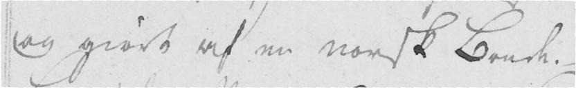og giort af en norsk Bonde.
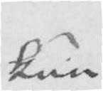kun
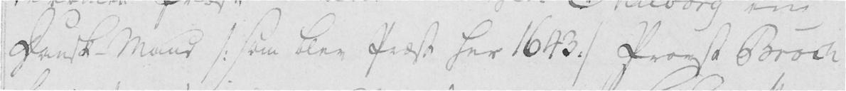Dansk Mand /: som blev Præst her 1643 :/ Provst Broch
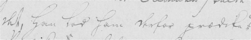det, Han lod ham derfor prædike;
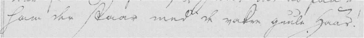ham der staar med de vakre guule Haar!
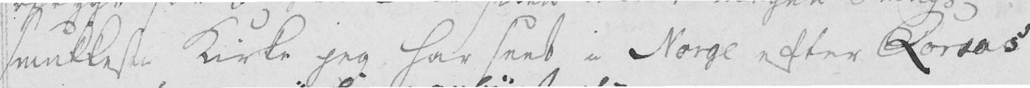smukkeste Kirke jeg har seet i Norge efter Røraas
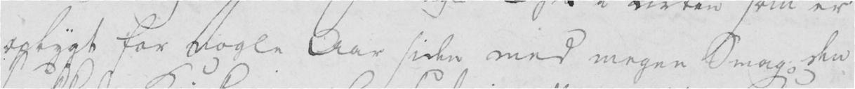opbygt for nogle Aar siden med megen Smag; den
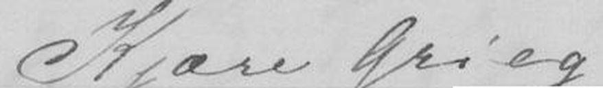Kjære Grieg,
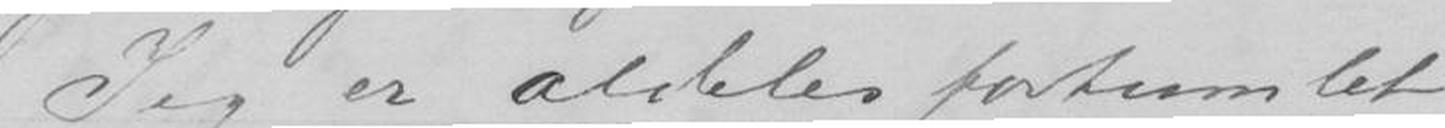Jeg er aldeles fortumlet
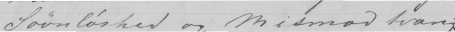Søvnløshed og Mismod tvang
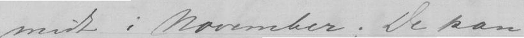midt i November; De kan
